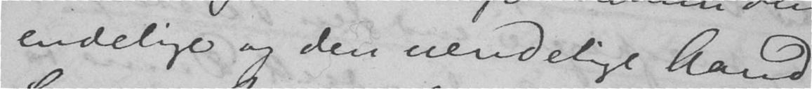endelige og den uendelige Aand.
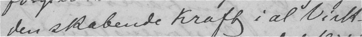den skabende Kraft i al Virk-
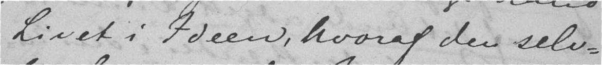Livet i Ideen, hvoraf den selv-
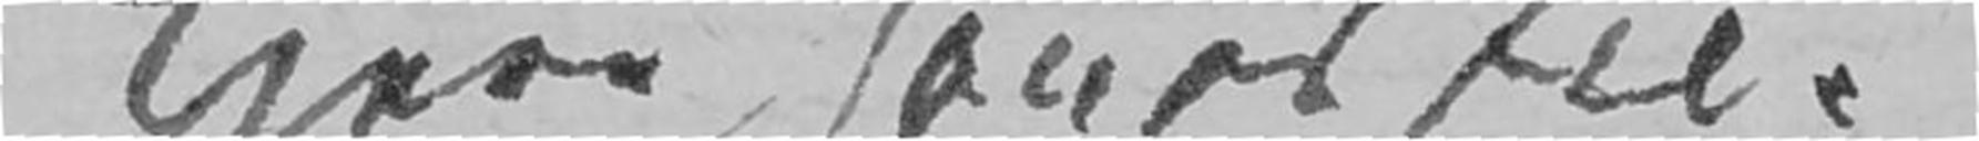Kjere Jonas Lie!
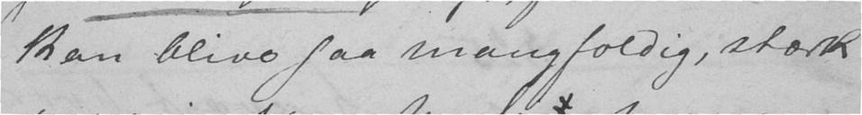kan blive saa mangfoldig, stærk
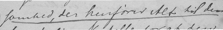somhed, der henfører Alt til den
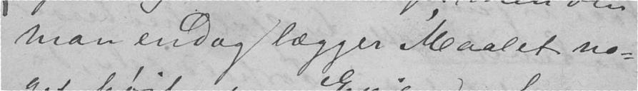man endog lægger Maalet no-
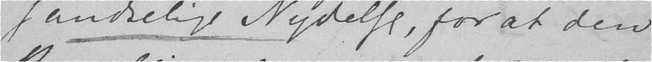sandselige Nydelse, for at den
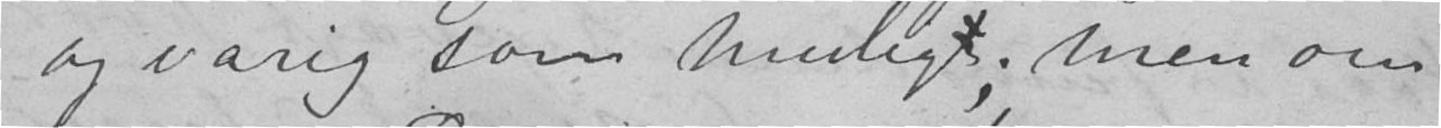og varig som muligt; men om
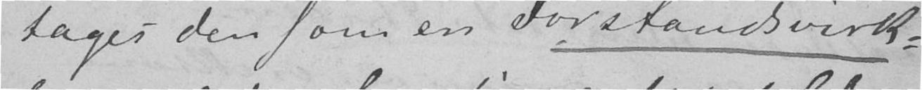tages den som en Forstandsvirk-
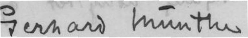Gerhard Munthe.
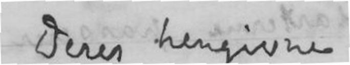Deres hengivne
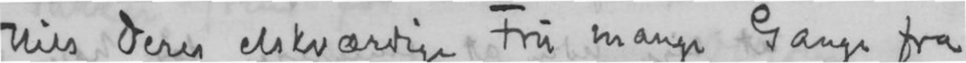Hils Deres elskværdige Fru mange Gange fra
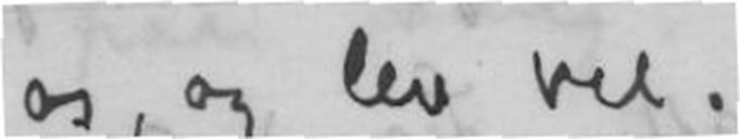os, og lev vel.
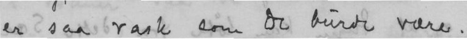er saa rask som De burde være.
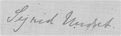Sigrid Undset.
